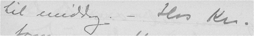til middag. - Hos Kr.
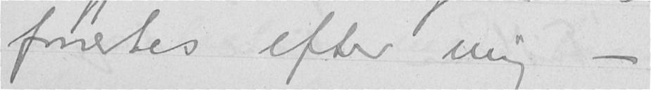fonertes efter mig -
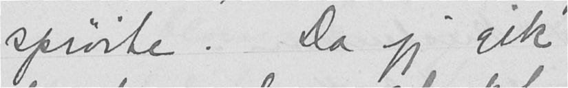sprøite. Da jeg gik
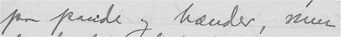paa pande og hænder, men
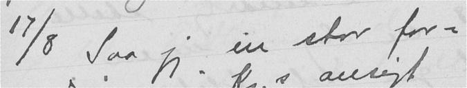17/8 Saa jeg en stor for-
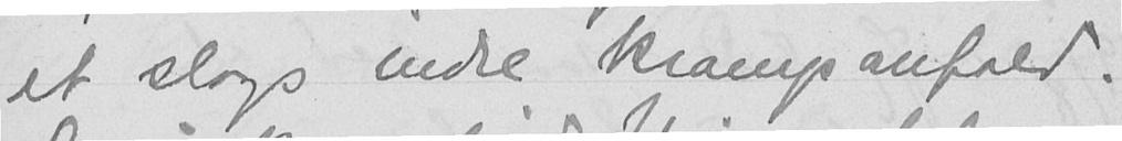et slags indre krampanfald.
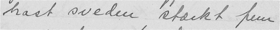brast sveden stærkt frem
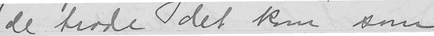de trode det kom som
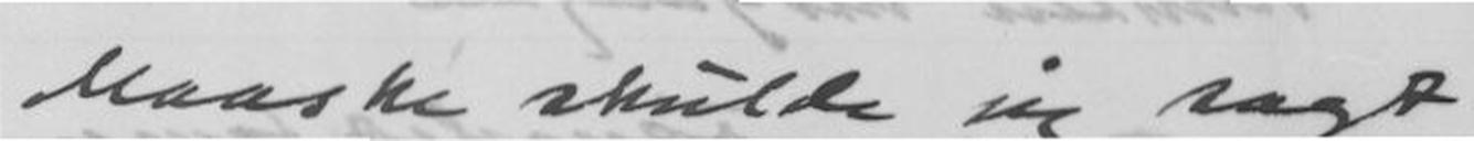Maaske skulde jeg sagt
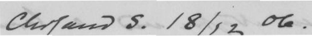Chrsand S. 18/12 06
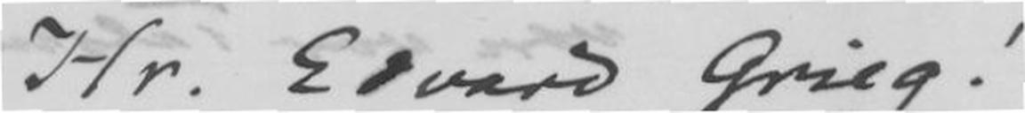Hr. Edvard Grieg!
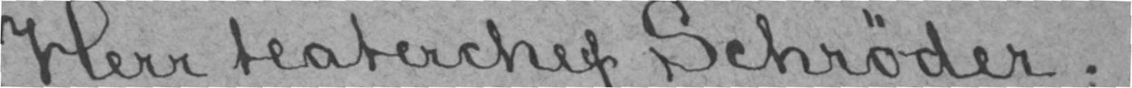Herr teaterchef Schrøder.
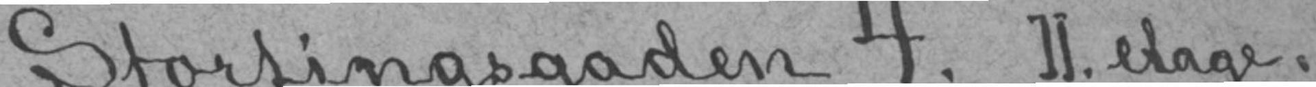Stortingsgaden 4. II. etage.
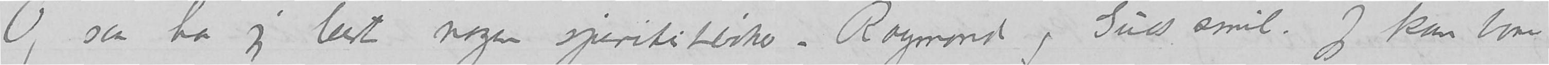Og saa har jeg læst nogen spiritistbøker . Raymond og Guds smil. Jeg kan bare
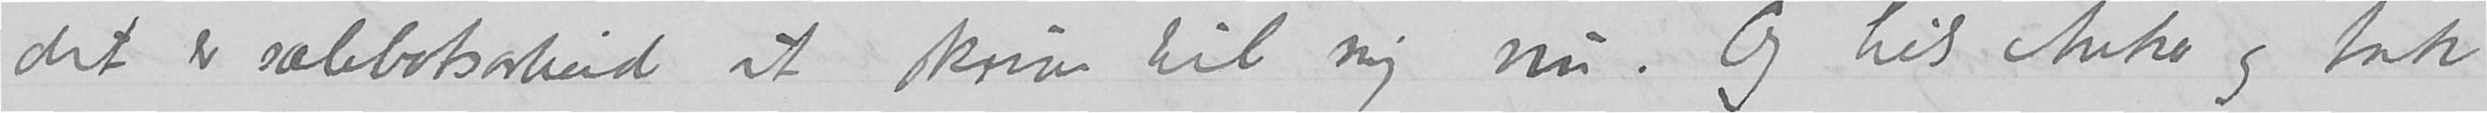det er sælebotsarbeid at skrive til mig nu.  Og hils Anker og tak
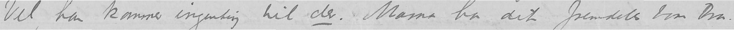Vel, han kommer ingenting til der. Mama har det fremdeles bare bra.
